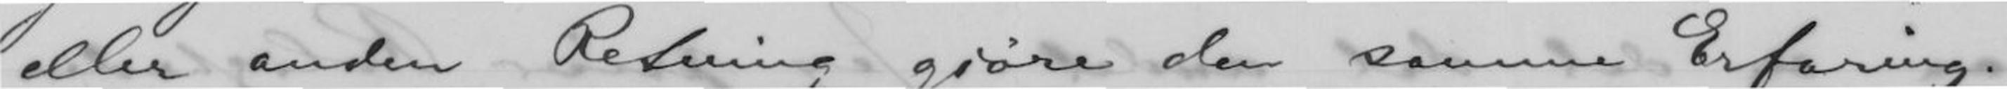eller anden Retning gjøre den samme Erfaring. -
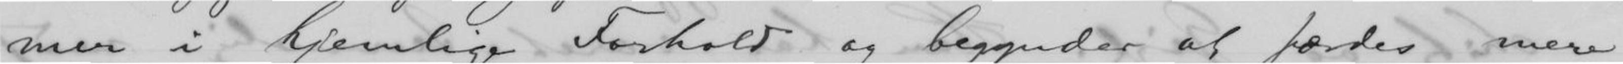mer i hjemlige Forhold og begynder at færdes mere
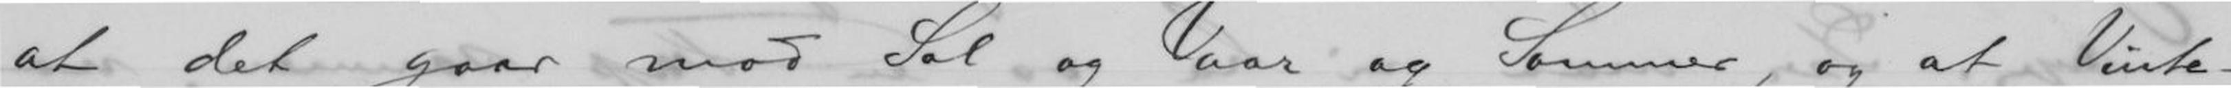at det gaar mod Sol og Vaar og Sommer, og at Vinte-
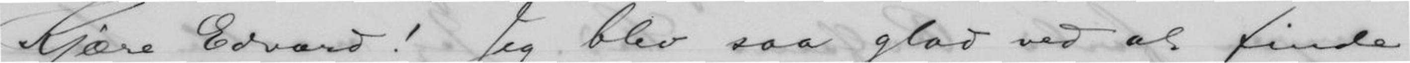Kjære Edvard! Jeg blev saa glad ved at finde
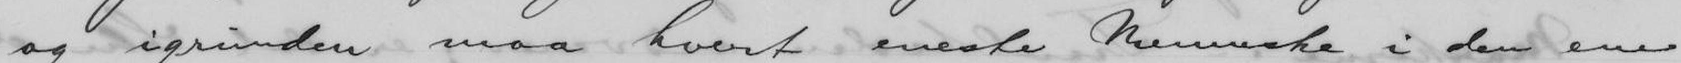og igrunden maa hvert eneste Menneske i den ene
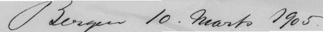Bergen 10. Marts 1905.
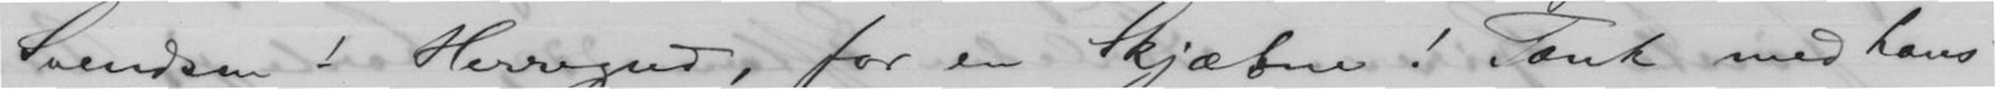Svendsen! Herregud, for en Skjæbne! Tænk med hans
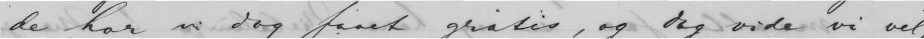de har vi dog faaet gratis, og dog vide vi vel,
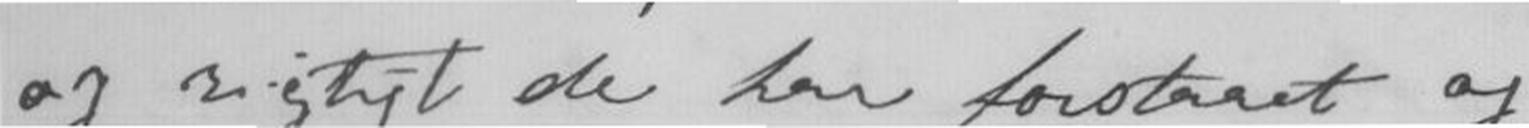og rigtigt de har forstaaet og
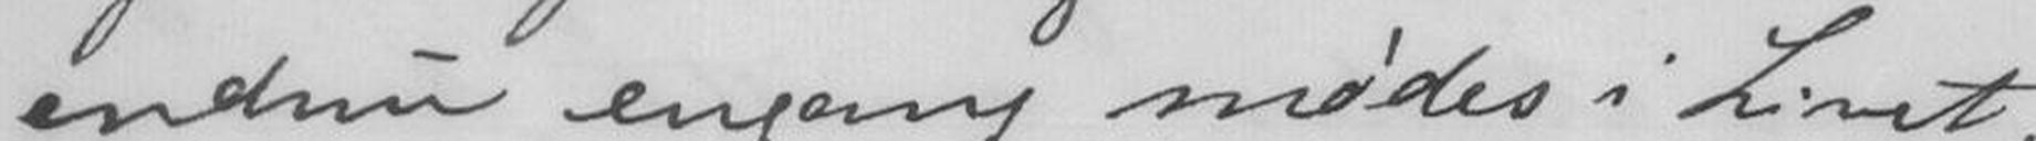endnu engang mødes i Livet,
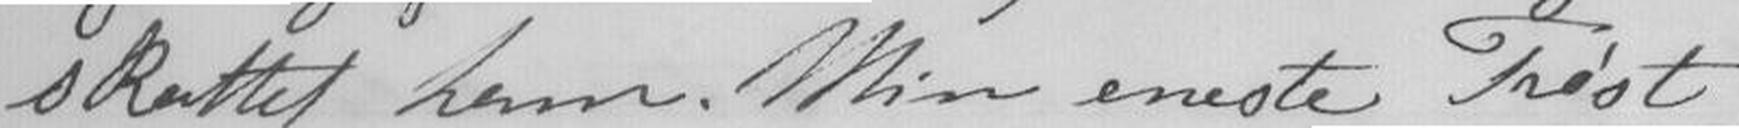skattet ham. Min eneste Trøst
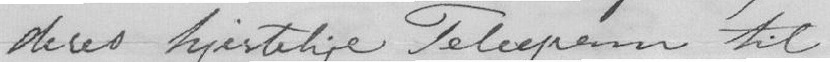deres hjertelige Telegram til
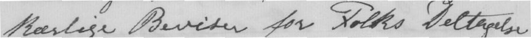Kærlige Beviser for Folks Deltagelse
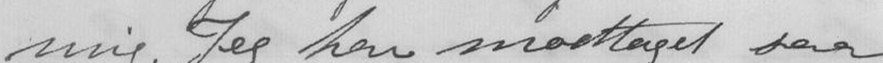mig. Jeg har modtaget saa
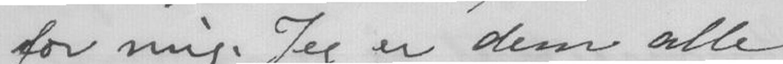for mig. Jeg er dem alle
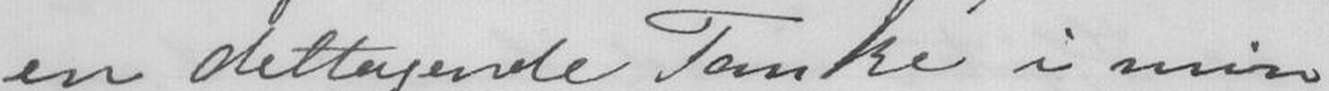en deltagende Tanke i min
